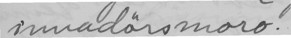innadørsmoro.
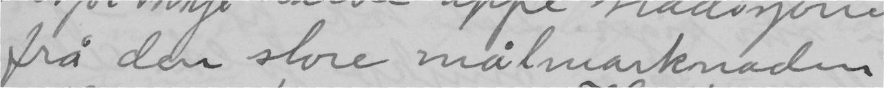frå den store målmarknaden
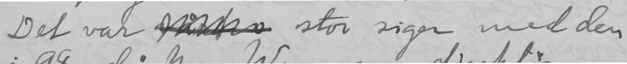Det var  stor siger med den
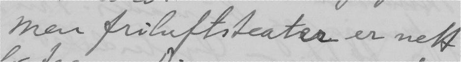Men friluftsteater er nett
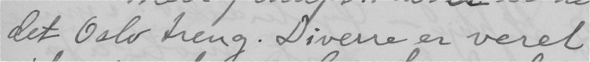det Oslo treng. Diverre er veret
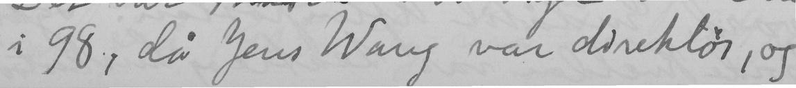i 98, då Jens Wang var direktör, og
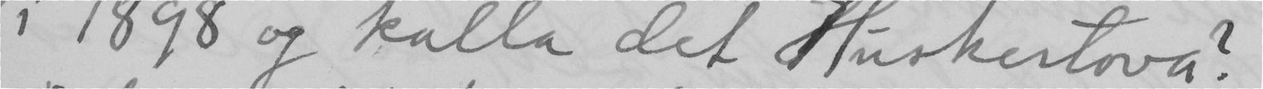i 1898 og kalla det Huskestova?
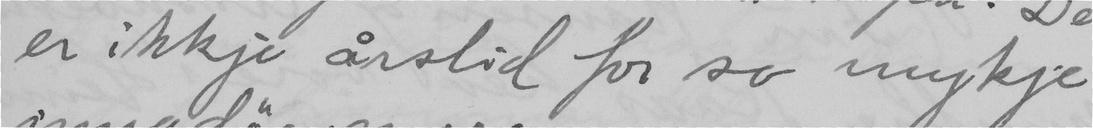er ikkje årstid for so mykje
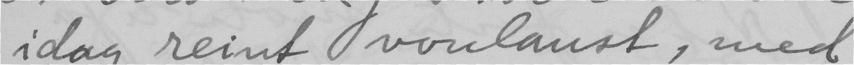idag reint vonlaust, med
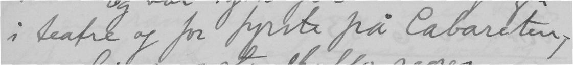i teatre og for fyrste på Cabareten,
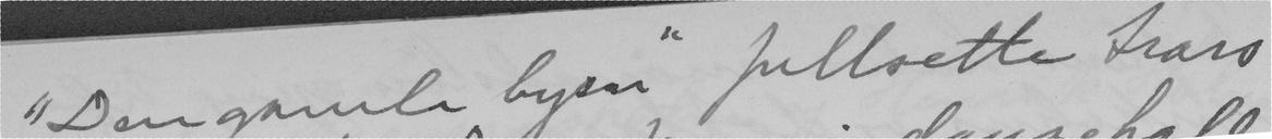"Den gamle byen" fullsette trass
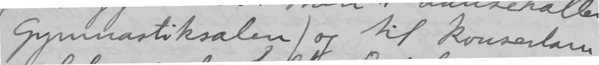(Gymnastiksalen) og til konsertane
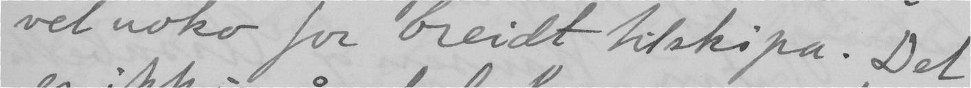vet noko for breidt tilskipa. Det
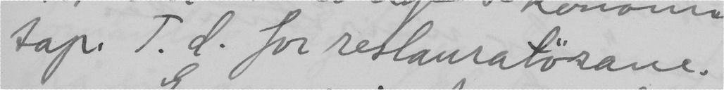tap T.d. for restaurantörane.
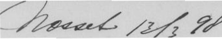Næsset 13/3 98
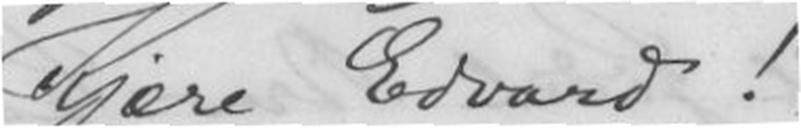Kjære Edvard!
